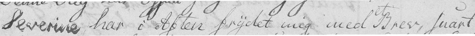Severine har i Aften frydet mig med et Brev, snart
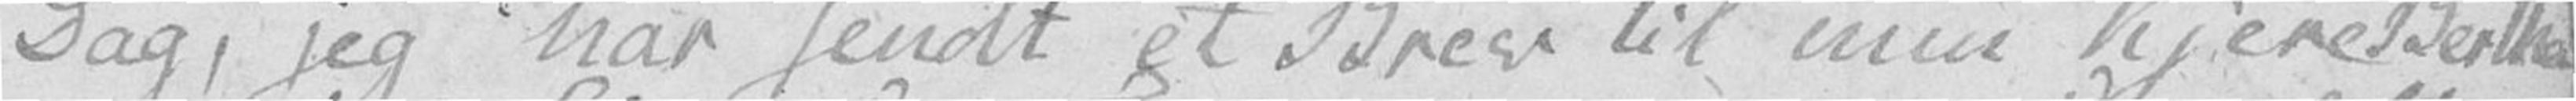Dag, jeg har sendt et Brev til min kjere Bertha
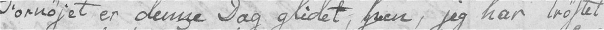Fornøjet er denne Dag glidet, hen, jeg har trøstet
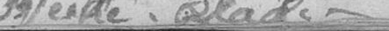Bjerke. Glad. –
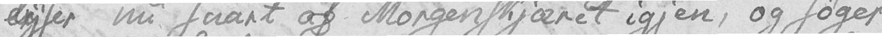lyser nu snart af Morgenskjæret igjen, og søger
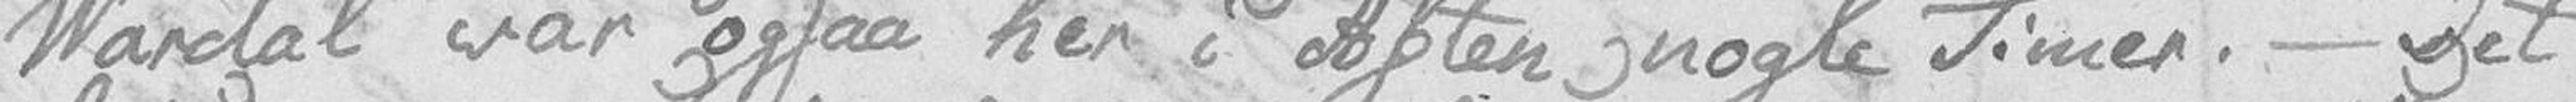Vardal var ogsaa her i Aften nogle Timer. – Det
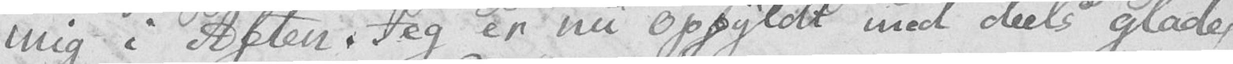mig i Aften. Jeg er nu opfyldt med deels glade,
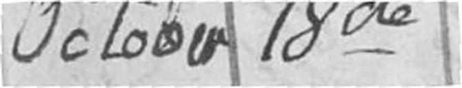October 18de
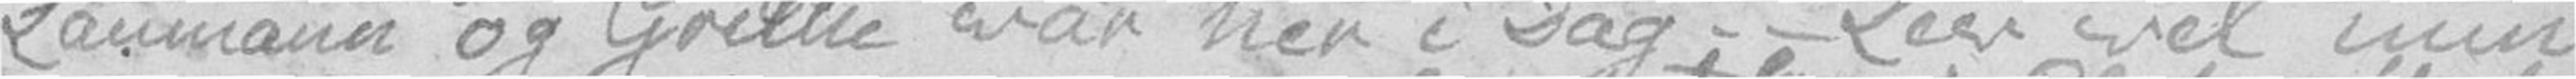Laumann og Grethe var her i Dag. – Lev vel min
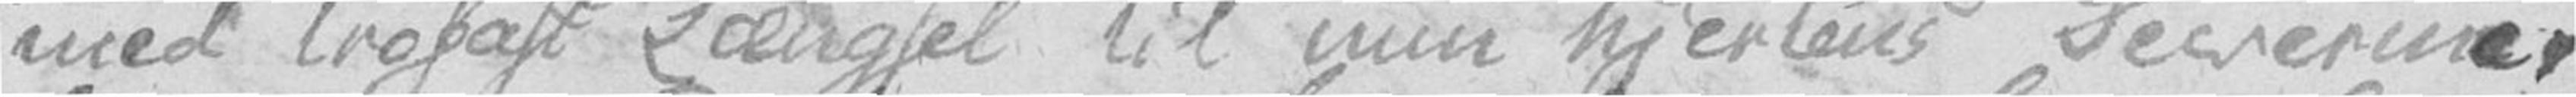med trofast Længsel til min hjertens Severine.
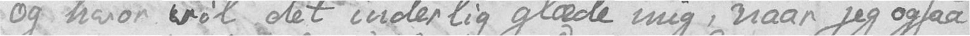og hvor vil det inderlig glæde mig, naar jeg ogsaa
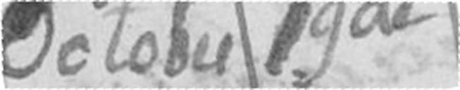October 19de
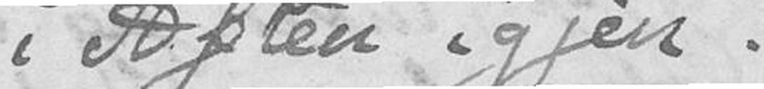i Aften igjen.
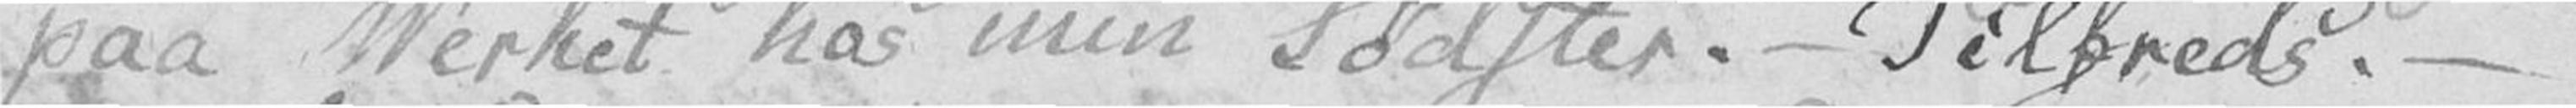paa Verket hos min Sødster. – Tilfreds. –
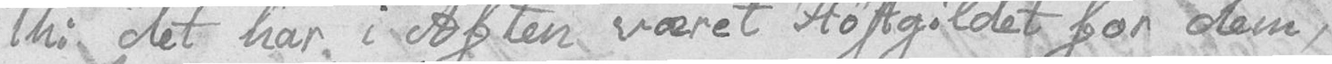thi det har i Aften været Høstgildet for dem,
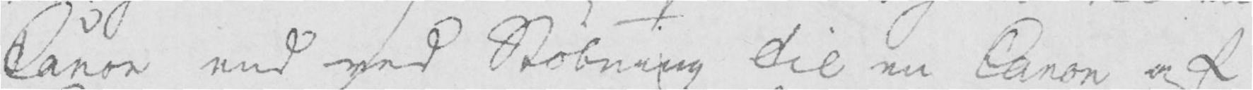Canon end ved Støbning til en Canon af
